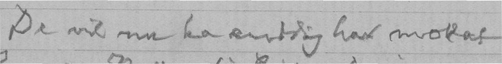De vil nu ha endelig har mottat
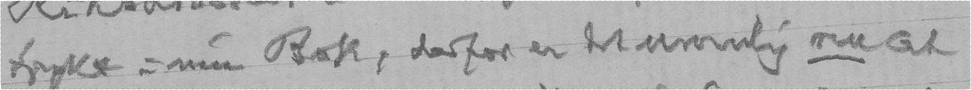trykt i min Bok, derfor er det umulig nu at
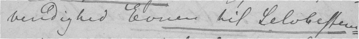vendighed Evnen til Selvbestem-
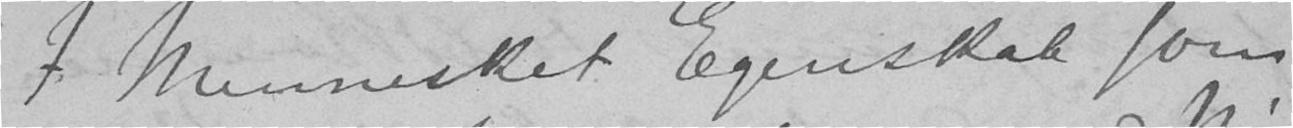I Menneskets Egenskab som
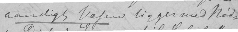aandigt Væsen ligger med Nød-
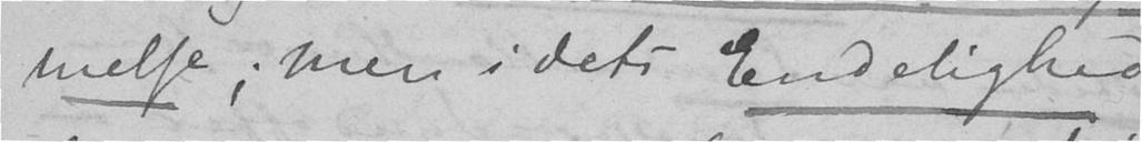melse; men i dets Endelighed
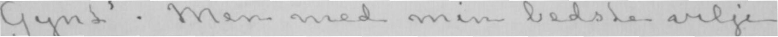Gynt». Men med min bedste vilje
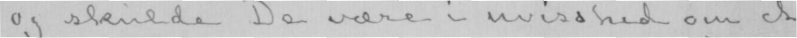og skulde De være i uvisshed om et
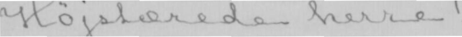Højstærede herre!
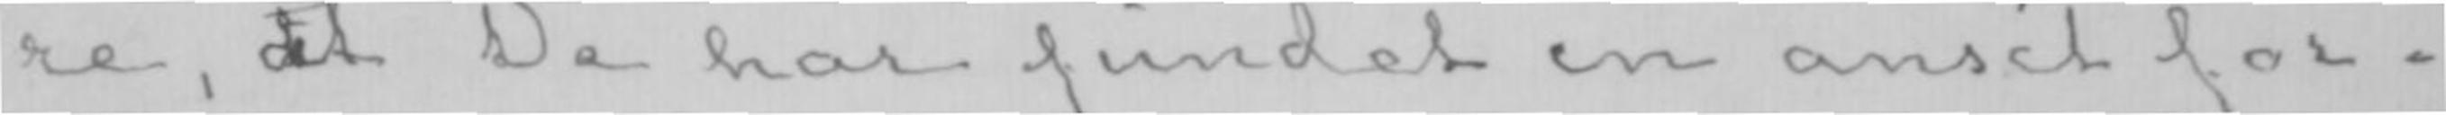re, at De har fundet en ansét for-
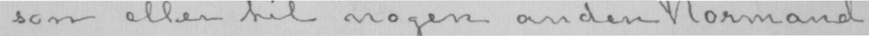son eller til nogen anden Normand.
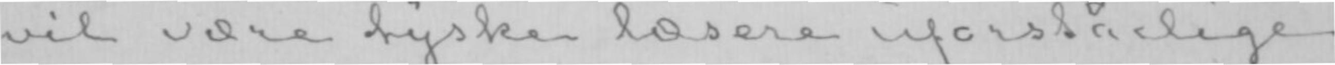vil være tyske læsere uforståelige;
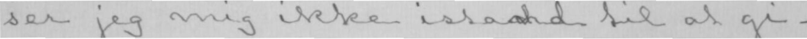ser jeg mig ikke istand til at gi-
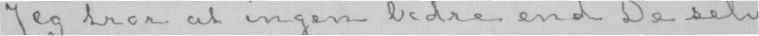Jeg tror at ingen bedre end De selv
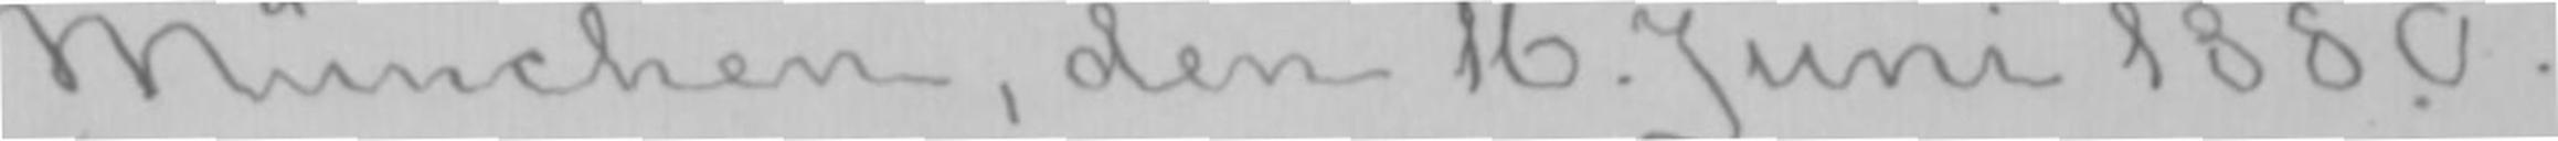München, den 16. Juni 1880.
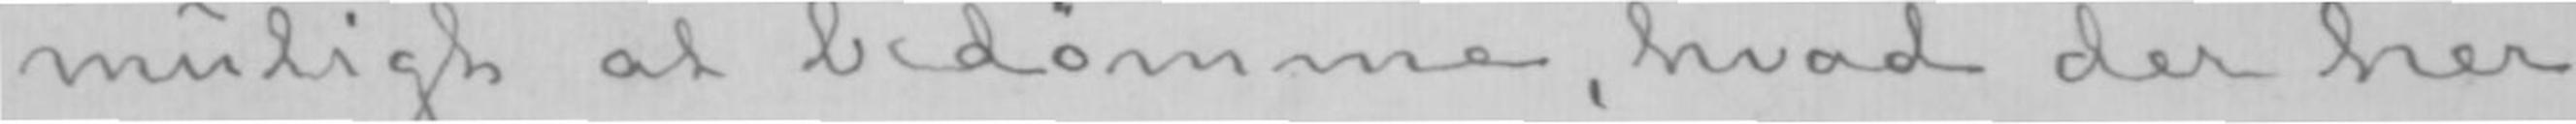muligt at bedømme, hvad der her
